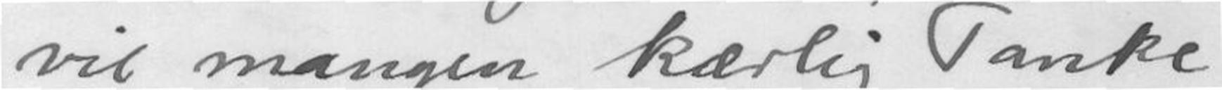vil mangen kærlig Tanke
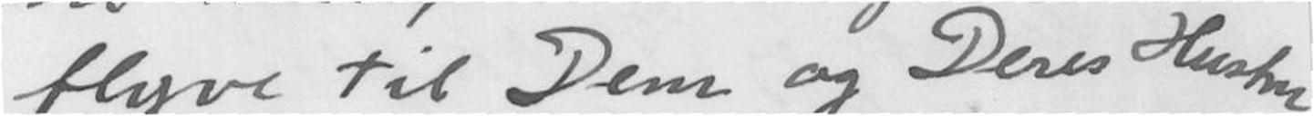flyve til Dem og Deres Hustru
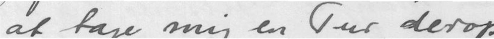at tage mig en Tur derop,
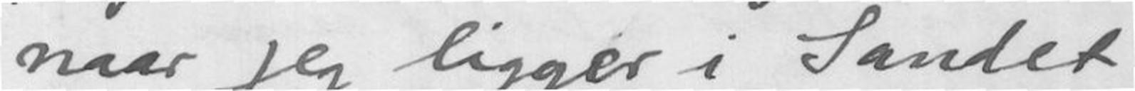naar jeg ligger i Sandet
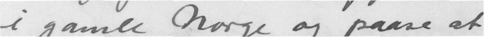i gamle Norge og paase at
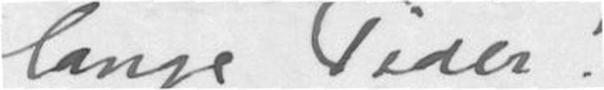lange Tider!
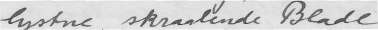lystne, skraalende Blade
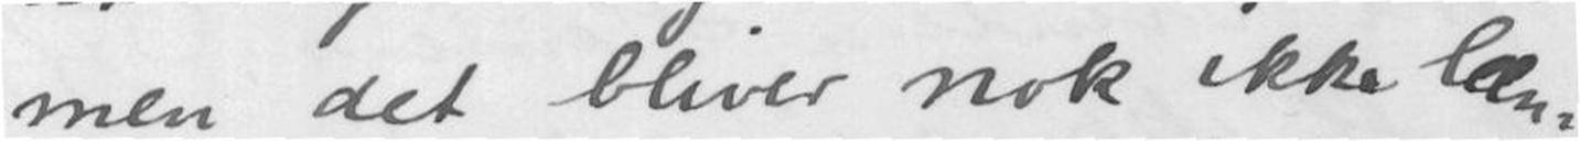men det bliver nok ikke læn-
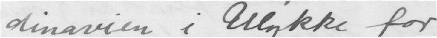dinavien i Ulykke for
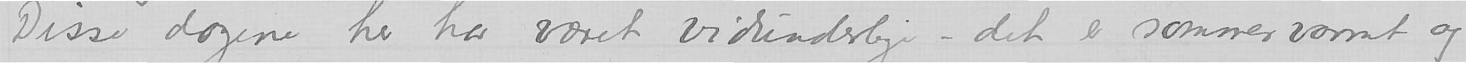Disse dagene her har været vidunderlige - det er sommervarmt og
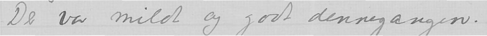Der var mildt og godt dennegangen.
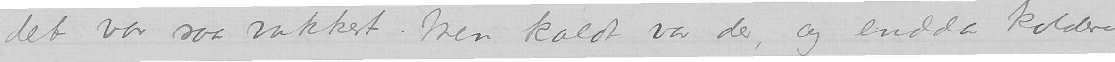det var saa vakkert. Men kaldt var der, og endda kaldere.
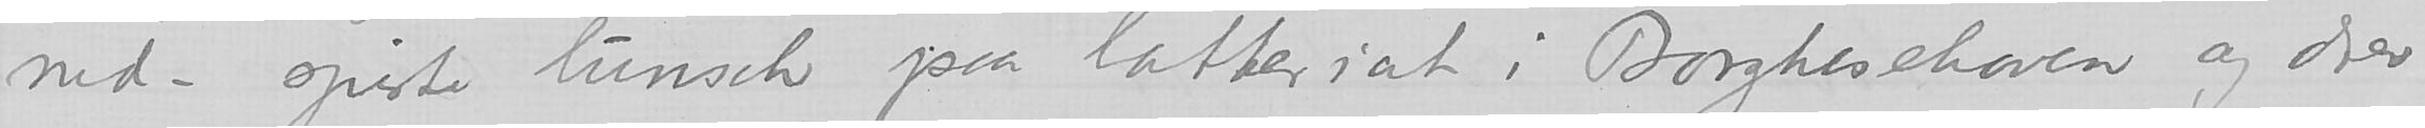ned - spiste lunsch paa latteriat i Borghesehaven og der
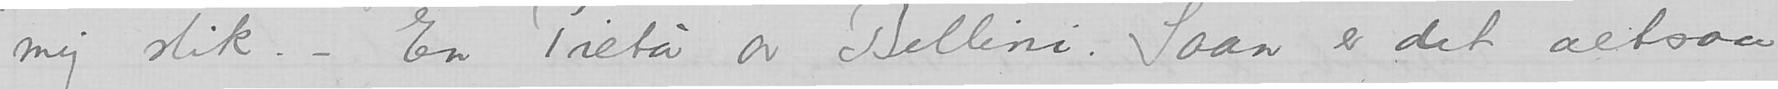mig slik. - En Pietá av Bellini. Saan er det altsaa
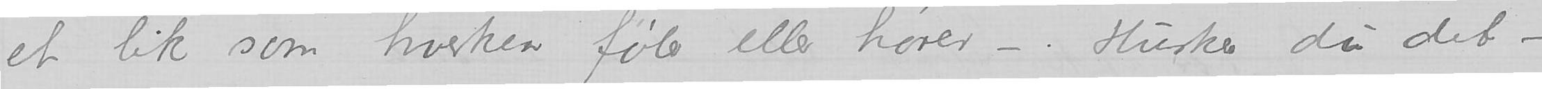et lik som hverken føler eller hører –. Husker du det
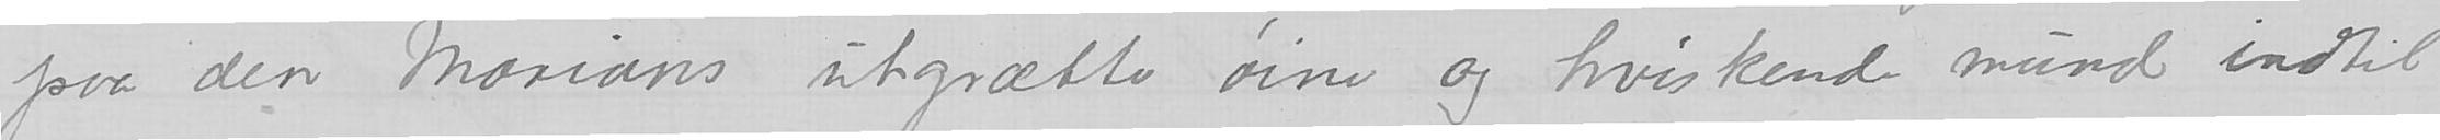paa den Marians utgrætte øine og hviskende mund indtil
